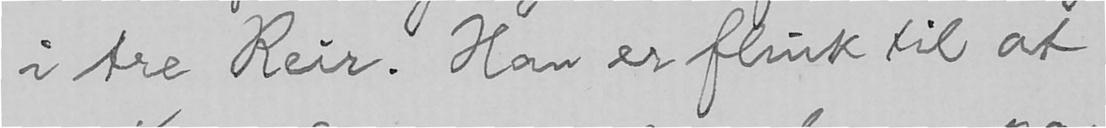i tre Reir. Han er flink til at
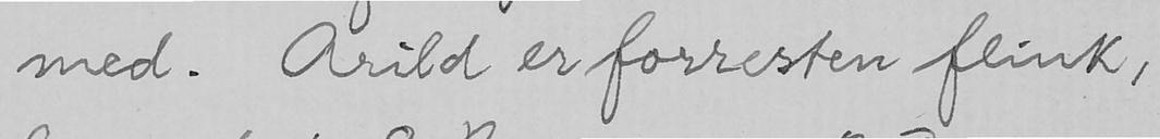med. Arild er forresten flink,
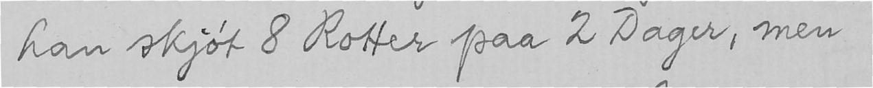han skjøt 8 Rotter paa 2 Dager, men
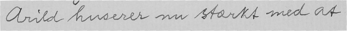Arild huserer nu stærkt med at
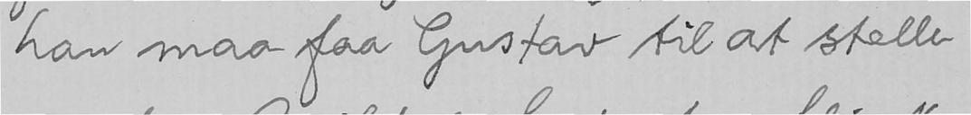han maa faa Gustav til at stelle
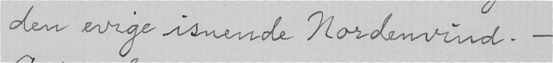den evige isnende Nordenvind. -
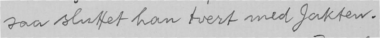saa sluttet han tvert med Jakten.
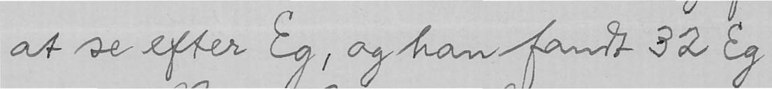at se efter Eg, og han fandt 32 Eg
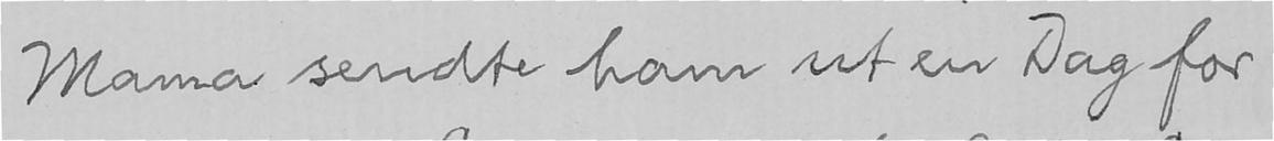Mama sendte ham ut en Dag for
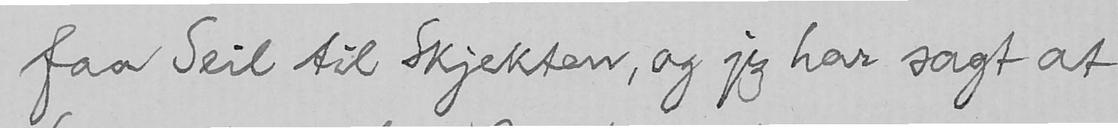faa Seil til Skjekten, og jeg har sagt at
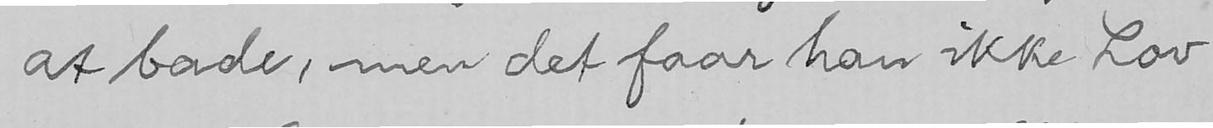at bade, men det faar han ikke Lov
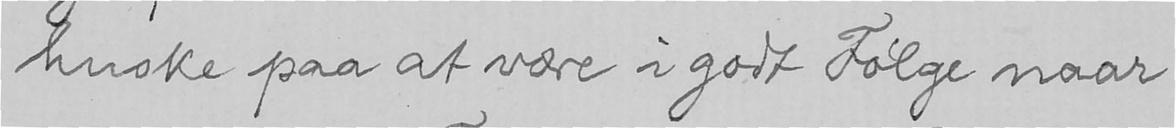huske paa at være i godt Følge naar
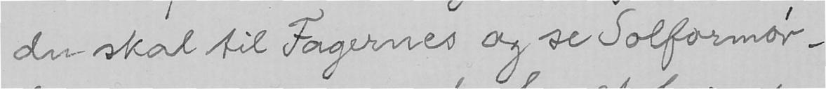du skal til Fagernes og se Solformør-
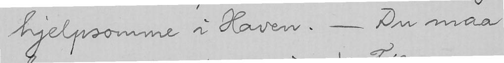hjelpsomme i Haven. - Du maa
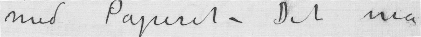med Papiret – Det må
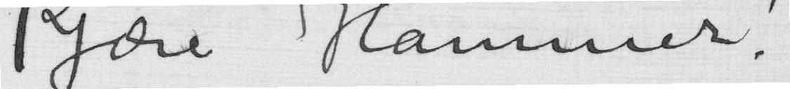Kjære Hammer!
